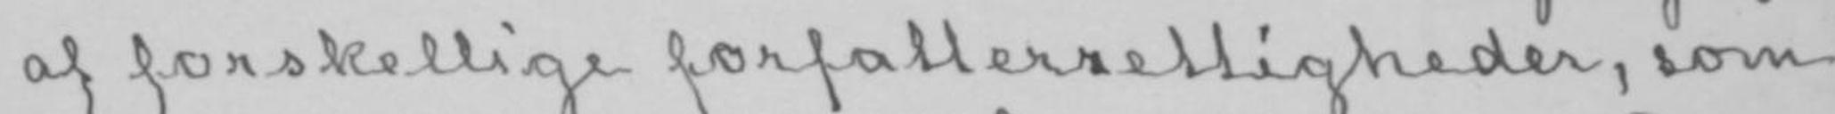af forskellige forfatterrettigheder, som
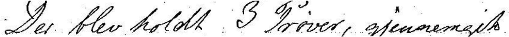Der blev holdt 3 Prøver, gjennemgik
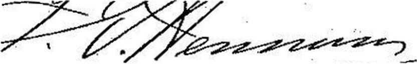J. E. Hennum
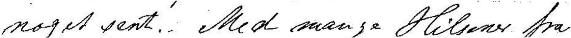noget sent. Med mange Hilsner fra
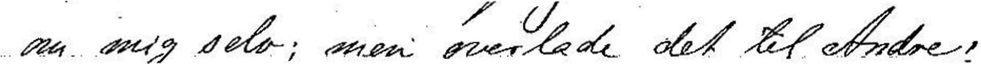om mig selv; men overlade det til Andre;
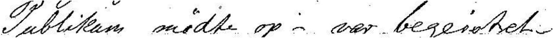Publikum mødte op - vær begeistret -
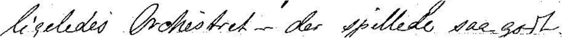ligeledes Orchestret - der spillede saa godt,
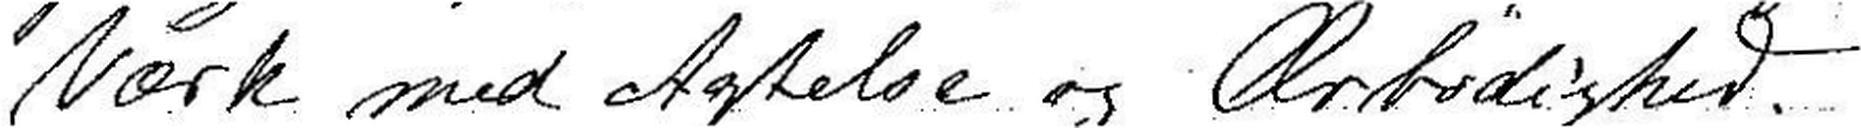Værk med Agtelse og Ærbødighed.
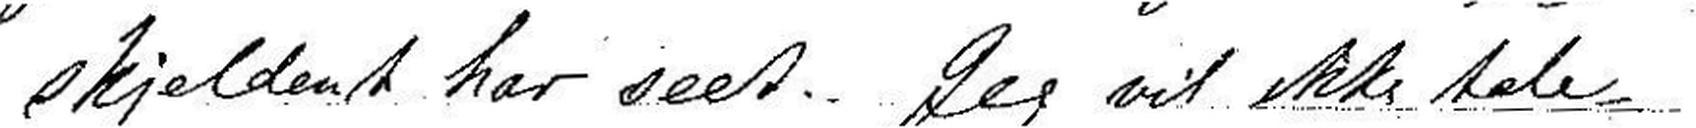skjeldent har seet. Jeg vil ikke tale
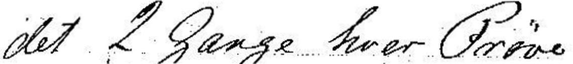det 2 Gange hver Prøve
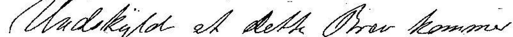Undskyld at dette Brev kommer
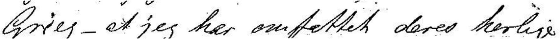Grieg - at jeg har omfattet deres herlige
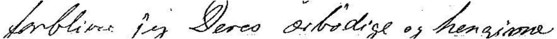forbliver jeg Deres ærbødige og hengivne
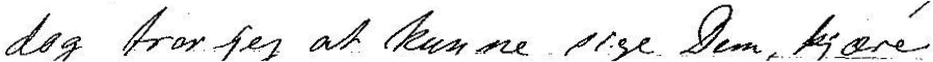dog tror jeg at kunne sige Dem, kjære
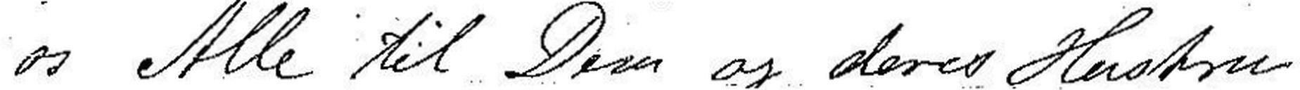os Alle til Dem og deres Hustru
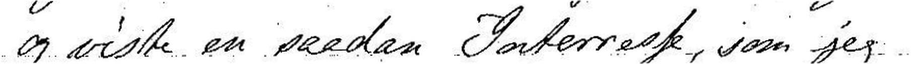og viste en saadan Interresse, som jeg
